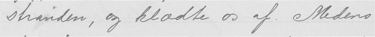stranden, og klædte os af. Medens
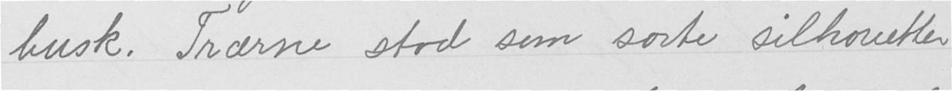busk. Trærne stod som sorte silhouetter
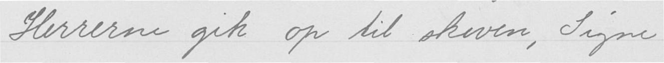Herrerne gik op til skoven, Signe
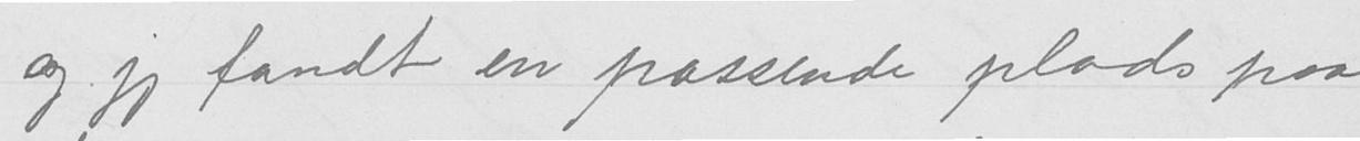og jeg fandt en passende plads paa
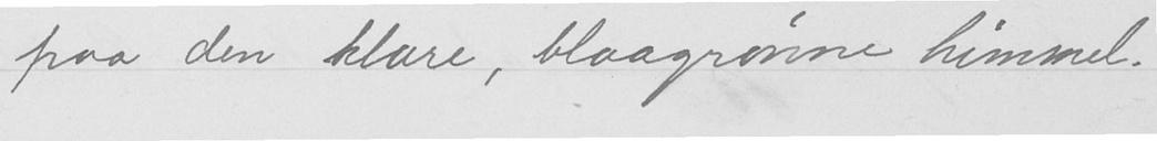paa den klare, blaagrønne himmel.
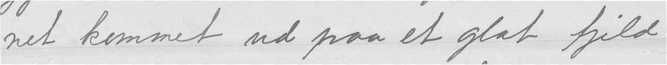net kommet ud paa et glat fjeld
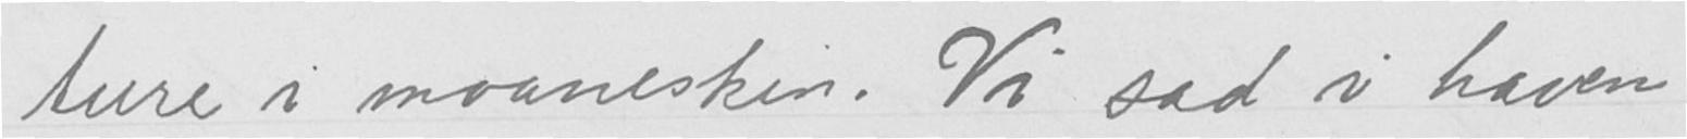ture i maaneskin. Vi sad i haven
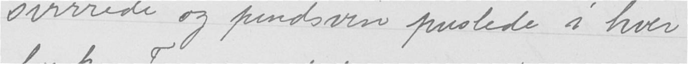svirrede og pindsvin puslede i hver
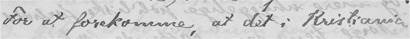For at forekomme, at det i Kristiania
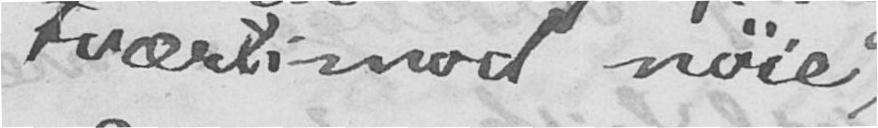tværtimod nøie
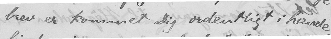brev er kommet Dig ordentligt i hænde,
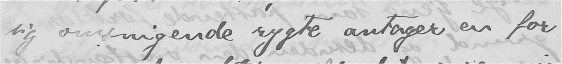sig omsvingende rygte antager en for
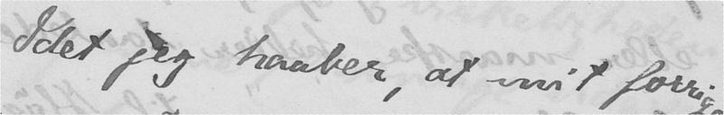Idet jeg haaber, at mit forrige
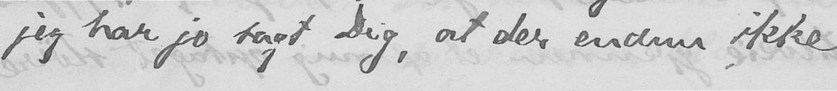jeg har jo sagt Dig, at der endnu ikke
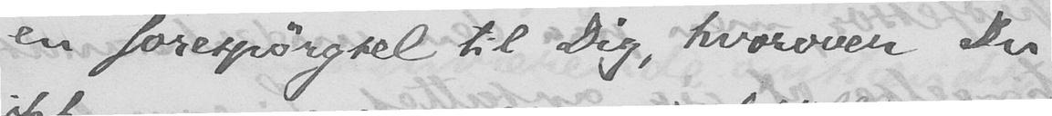en forespørgsel til Dig, hvorover Du
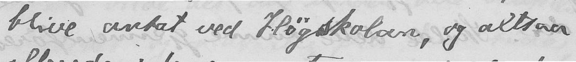blive ansat ved Høgskolan, og altsaa
